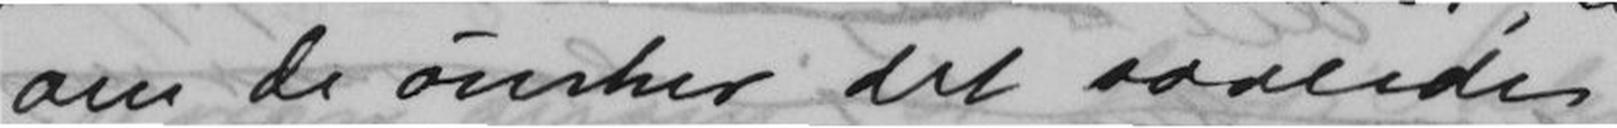om De ønsker det saaledes
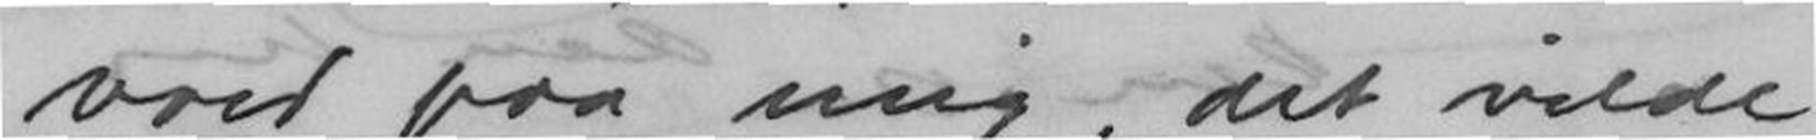vred paa mig, det vilde
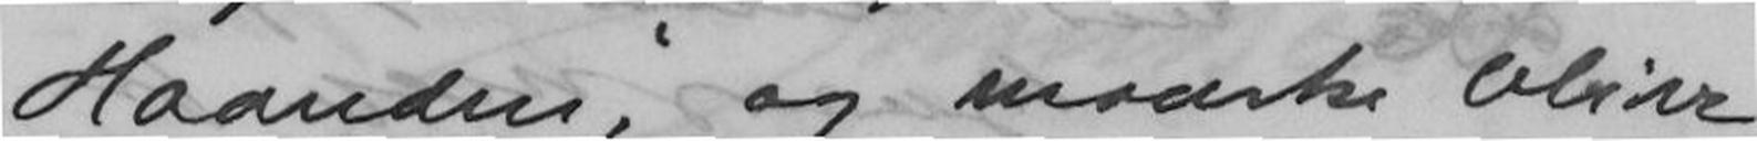Haanden, og maaske bliver
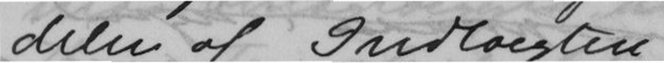delen af Indtægten.
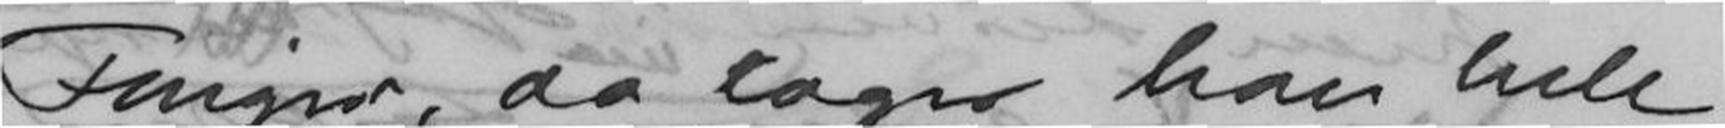Finger, da tager han hele
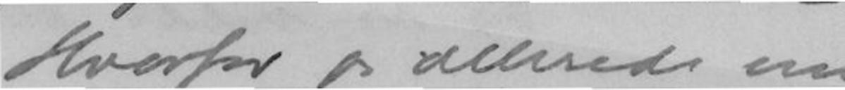Hvorfor jeg allerede nu
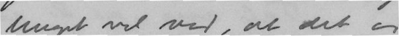meget vel ved, at det er
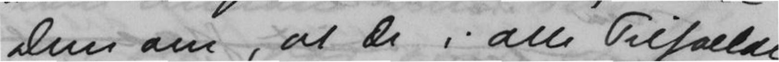Dem om, at De i alle Tilfælde
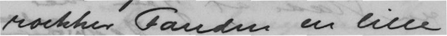rækker Fanden en lille
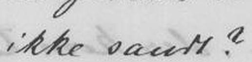ikke sandt?
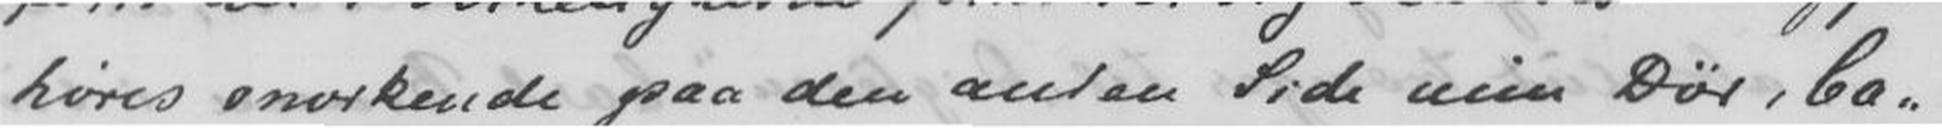høres snorkende paa den anden Side min Dør, Ca-
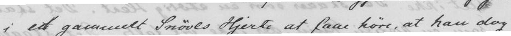i et gammelt Snøvls Hjerte at faae høre, at han dog
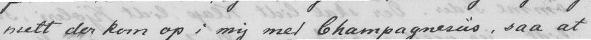melt der kom op i mig med Champagnesus, saa at
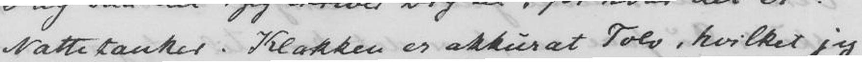Nattetanker. Klokken er akkurat Tolv, hvilket jeg
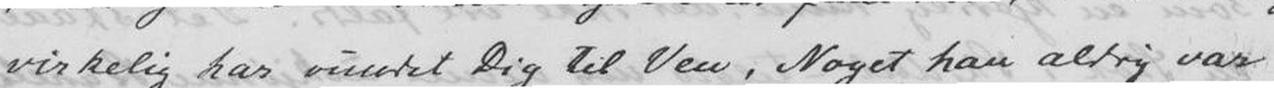virkelig har vundet Dig til Ven. Noget han aldrig var
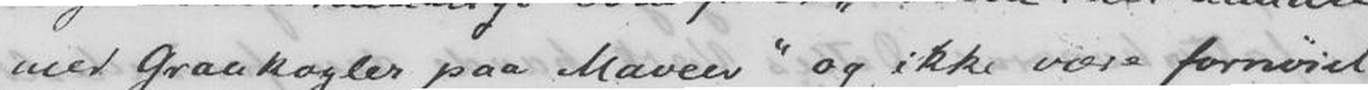med Grankogler paa Maven og ikke være fornøiet
